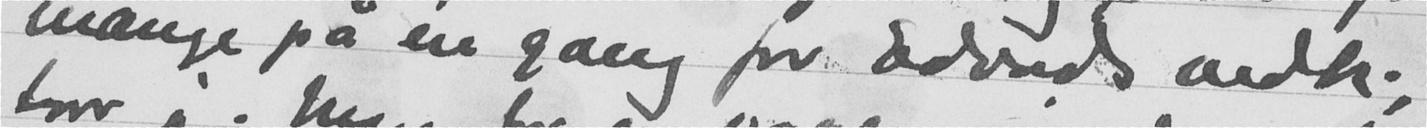mange på en gang for Edvards vedk.
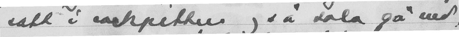satt i cockpitten og så sola gå ned,
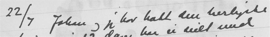22/7 Johan og jeg har hatt den herligste
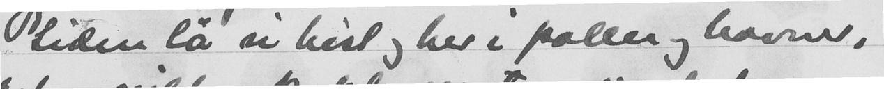Siden lå vi hist og her i poller og havner,
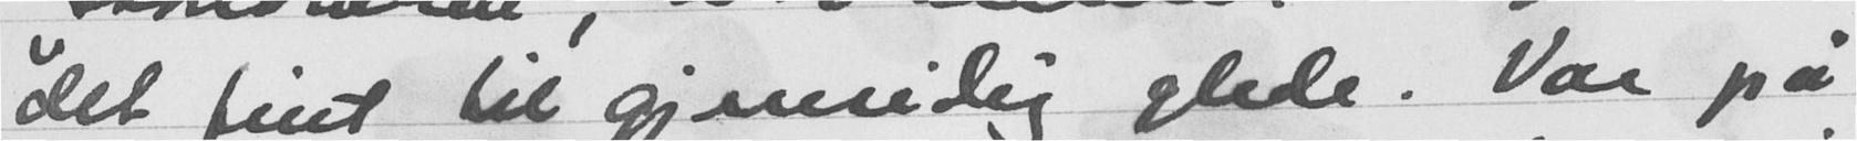det fint til gjensidig glede. Var på
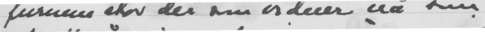furuene står der som videre nå som
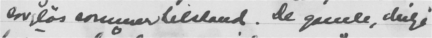sorgløs sommertilstand. De gamle, deilige
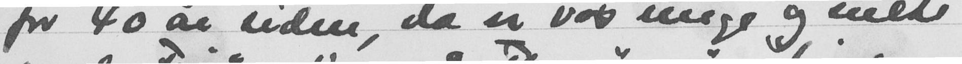for 40 år siden, da vi var unge og vilde
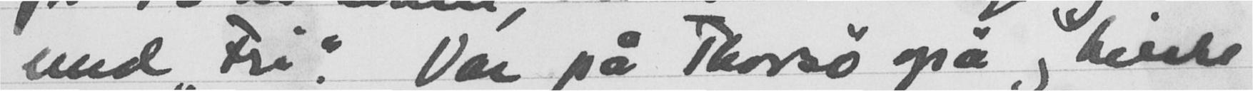med "Fri". Var på Thorsø også og hilste
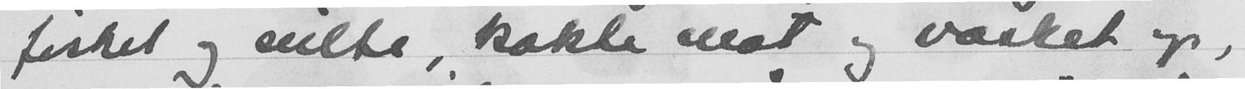fisket og seilte, kokte mat og vasket op,
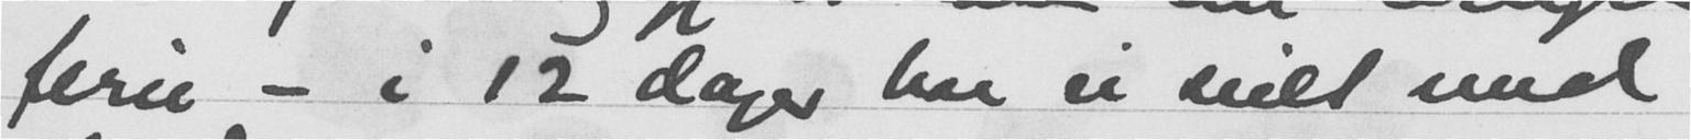ferie - i 12 dager har vi seilt med
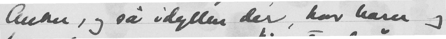Anker, og så idyllen der, hvor barn og
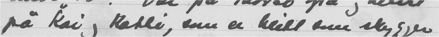på Kai og Katti, som er blitt som skygger
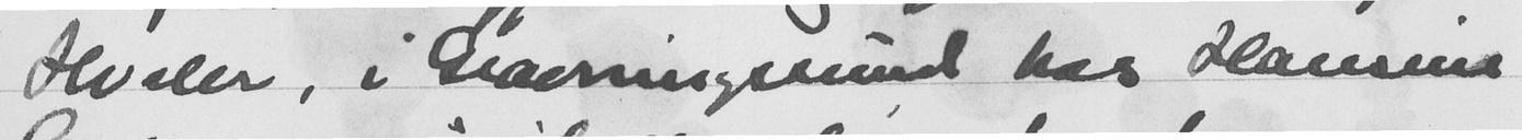Hvaler, i Guvningssund hos Hansine
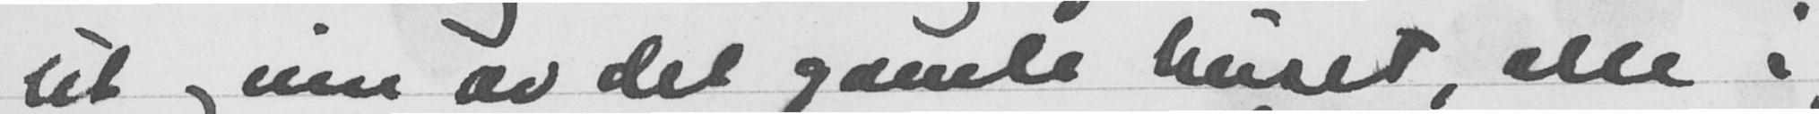ut og inn av det gamle huset, alle i
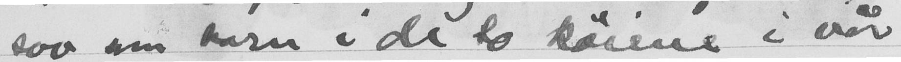sov som barn i de to køiene i vår
